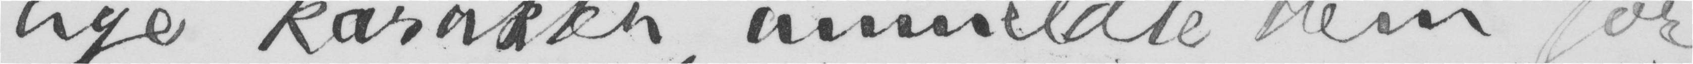lige karakter, anmeldte dem for
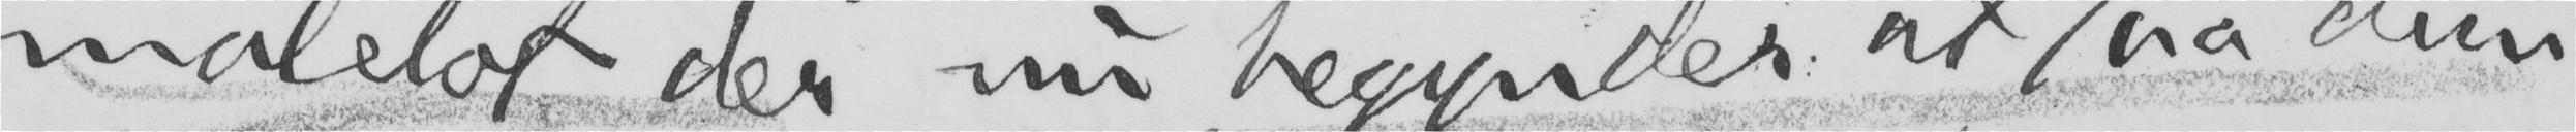matelot, der nu begynder at faa dun
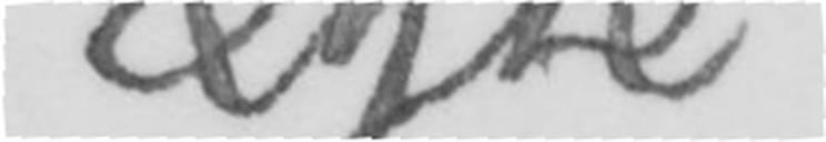ægte
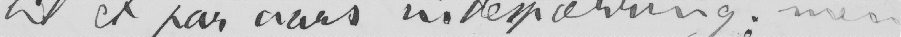til et par aars indespærring; men
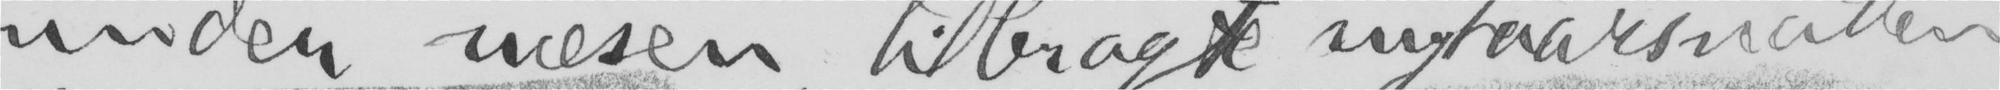under næsen, tilbragte nytaarsnatten
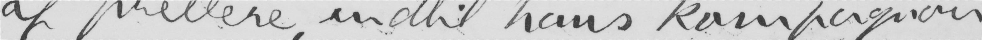af prellere, indtil hans kompagnon,
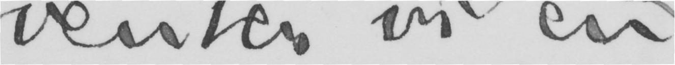venter vi en
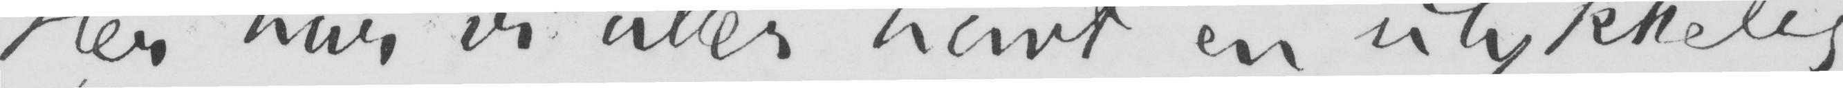Her har vi atter havt en ulykkelig
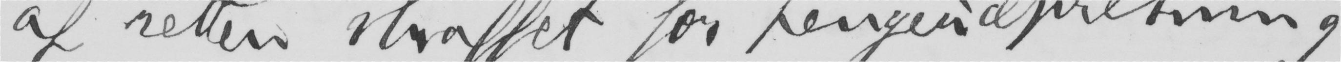af retten straffet for pengeudpresning
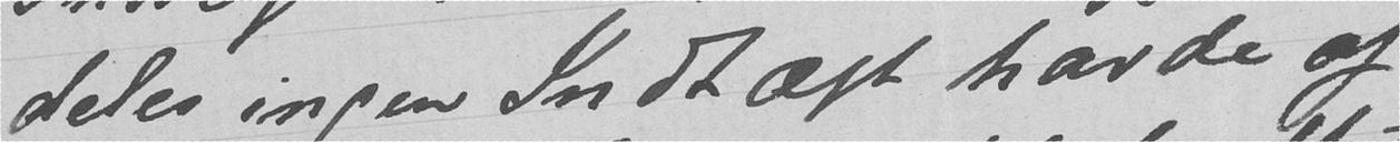deles ingen Indtægt havde af
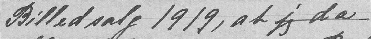Billedsalg 1919, at jeg da
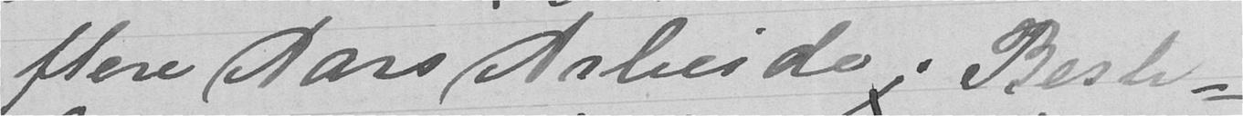flere Aars Arbeide i Besti-
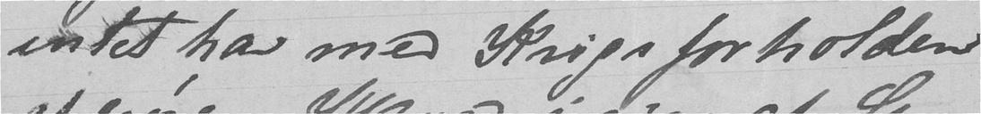intet har med Krigsforholdene
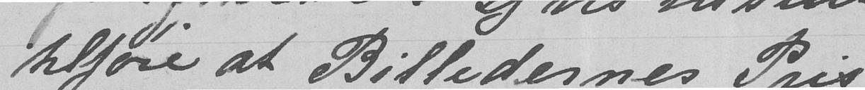tilføie at Billedernes Pris
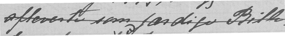afleverte som færdige Bille-
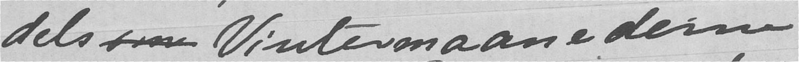dels om Vintermaanederne
